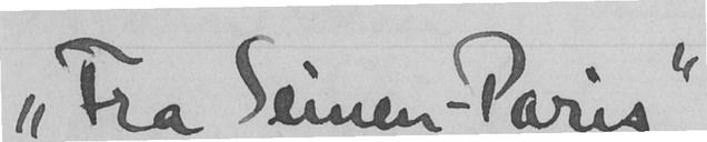"Fra Seinen - Paris"
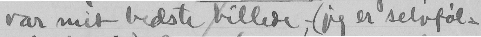var mit bedste billede, (jeg er selvføl-
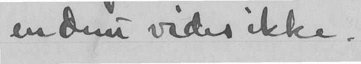endnu vedes ikke.
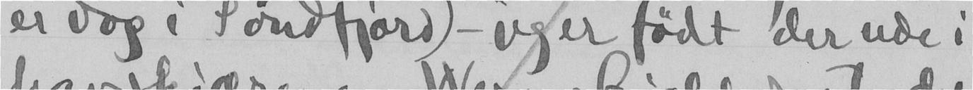er dog i Søndfjord) - jeg er født der ude i
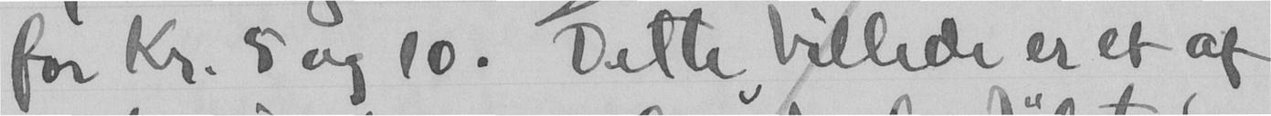for Kr. 5 og 10. Dette billede er et af
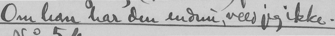Om hare har den endnu, veed jeg ikke.
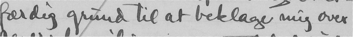færdig grund til at beklage mig over
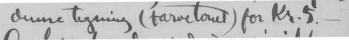denne tegning (farvetonet) for Kr.5. -
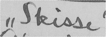"Skisse"
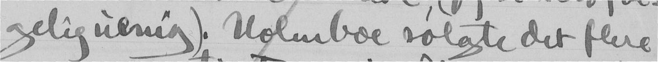gelig uenig). Holmboe solgte det flere
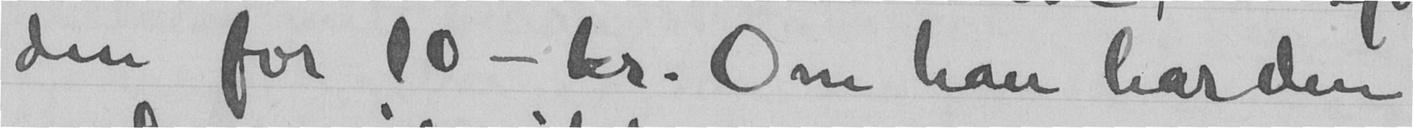den for 10 - kr. Om han har den
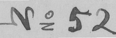No 52
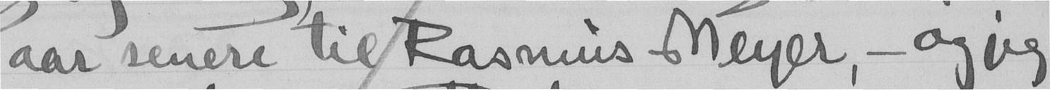aar senere til Rasmus Meyer, - og jeg
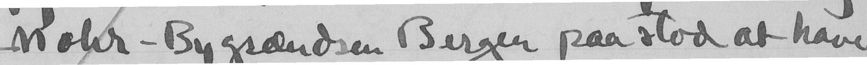Mohr-Bygsændsen Bergen paastod at have
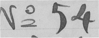No 54
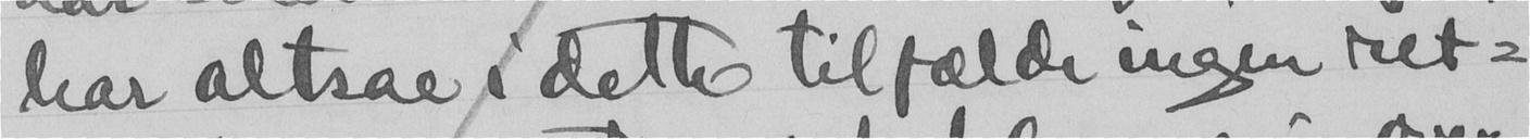har altsaa i dette tilfælde ingen ret-
